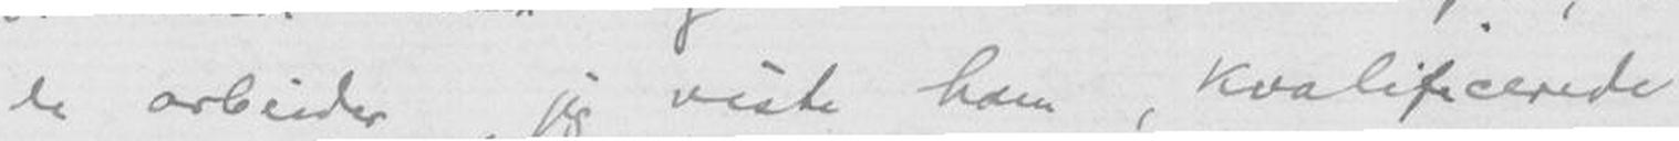de arbeider jeg viste ham, kvalificerede
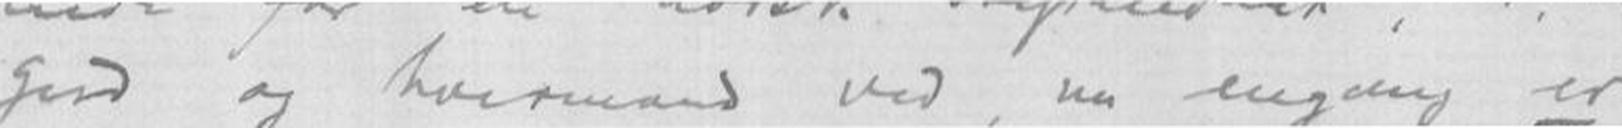Gud og hvermand ved, nu engang er
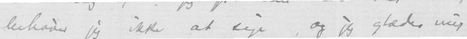behøves jeg ikke at sige og jeg glæder mig
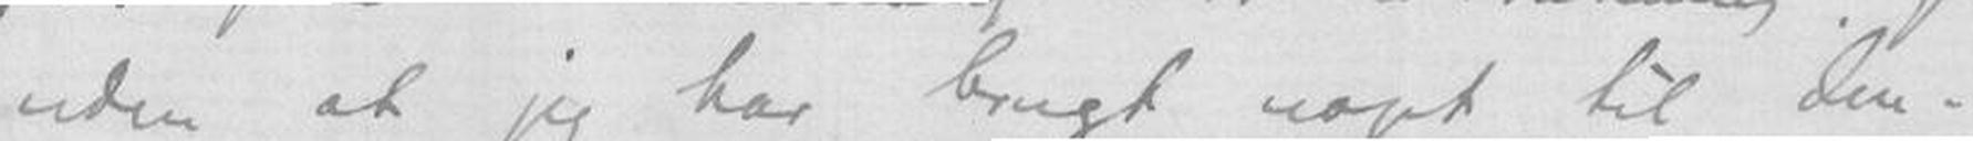uden at jeg har brugt noget til den-
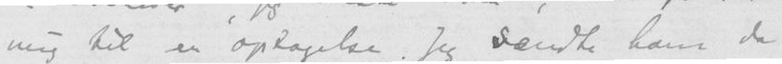mig til en optagelse. Jeg sændte ham da
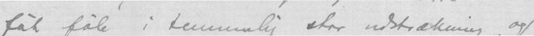fåt føle i temmelig stor udstrækning og
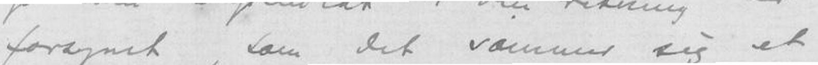forægnet, som det sømmer sig et
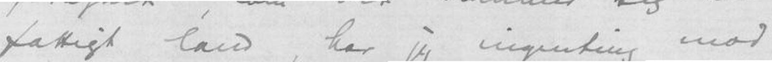fattigt land, har jeg ingenting mod
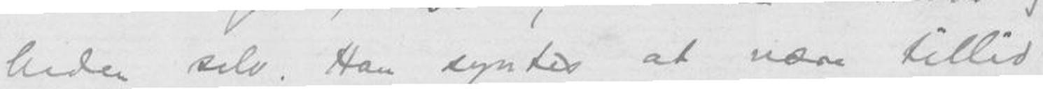heden selv. Han syntes at nære tillid
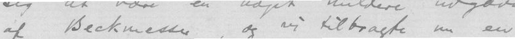af Beckmesser, og vi tilbragte nu en
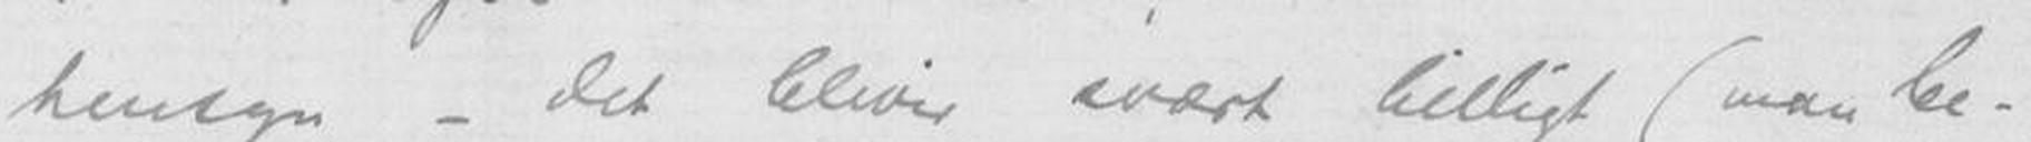hensyn - det bliver svært billigt (man be-
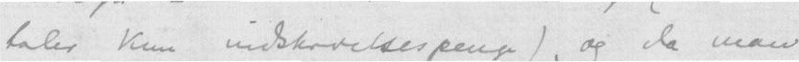taler kun indskrivelsespenge), og da man
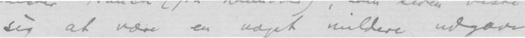sig at være en noget mildere udgave
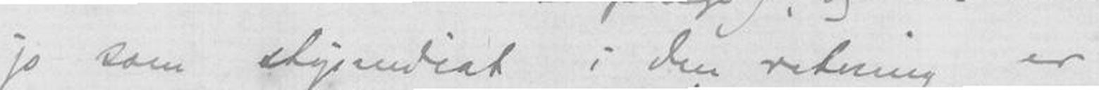jo som stipendiat i den retning er
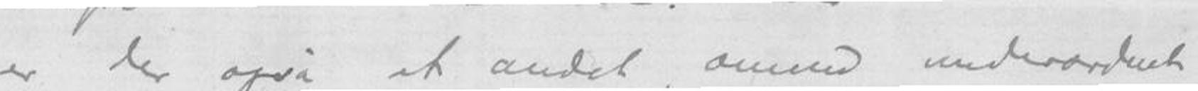er der også et andet, omend underordnet
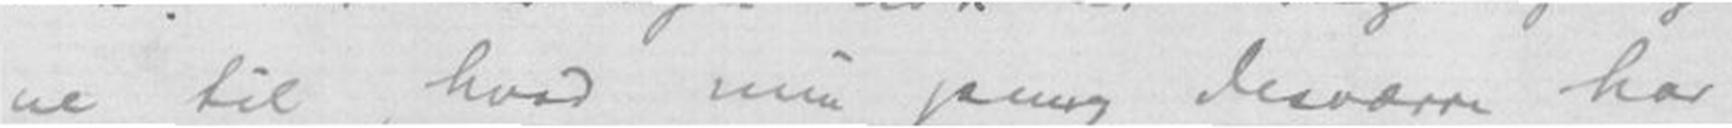ne til, hvad min pung desværre har
